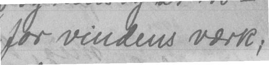for vindens værk;
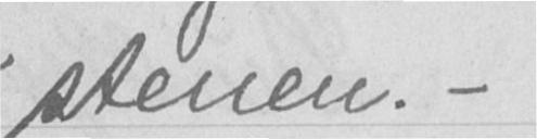stenen. -
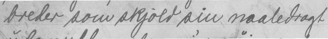breder som skjold sin naaledragt
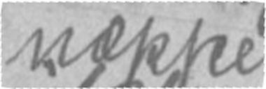næppe
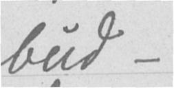bud -
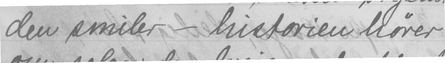den smiler - historien hører
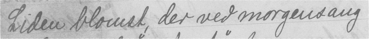Liden blomst der ved morgensang
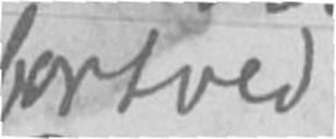bortved
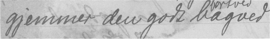gjemmer den godt bagved
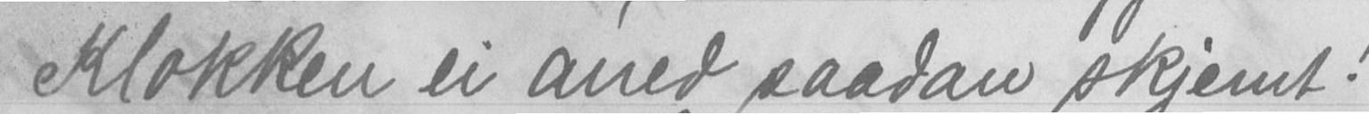Klokken ei aned saadan skjemt!
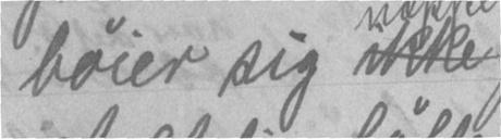bøier sig ikke
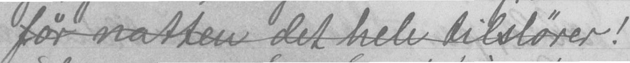før natten det hele tilslører!
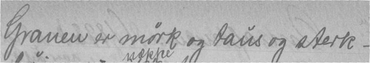Granen er mørk og taus og sterk -
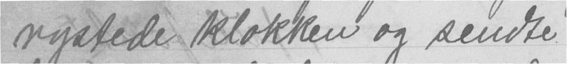rystede klokken og sendte
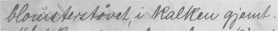blomsterstøvet, i kalken gjemt.
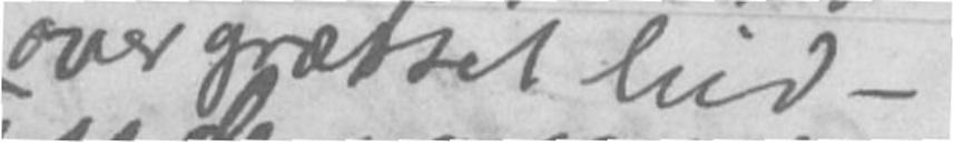over græsset hid -
